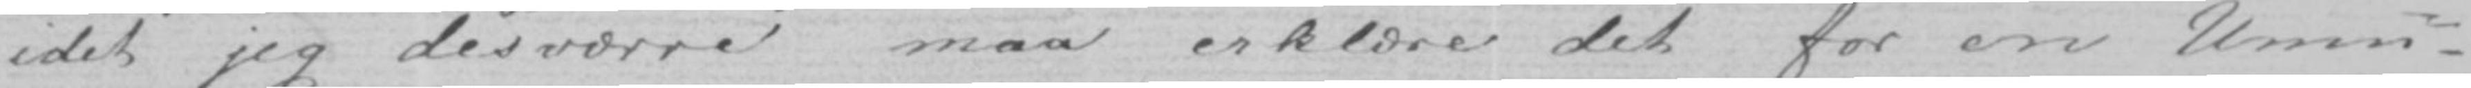idet jeg desværre maa erklære det for en Umu-
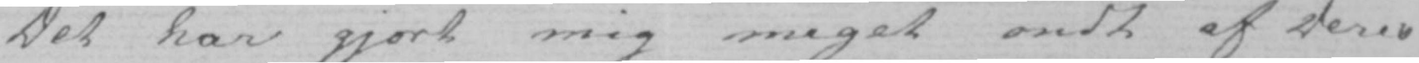Det har gjort mig meget ondt af Deres
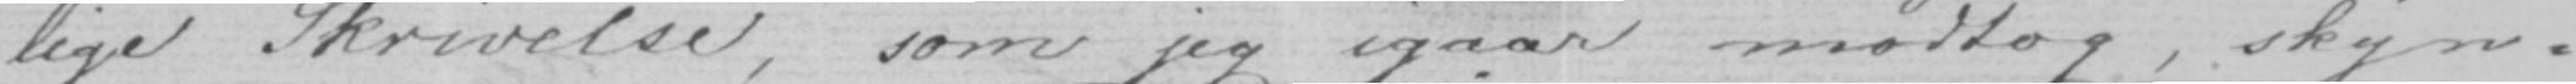lige Skrivelse, som jeg igaar modtog, skyn-
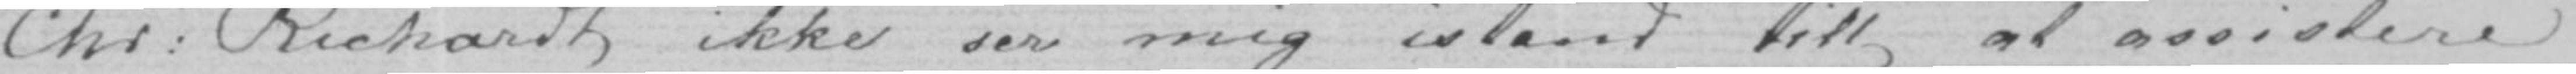Chr: Richardt ikke ser mig istand till at assistere
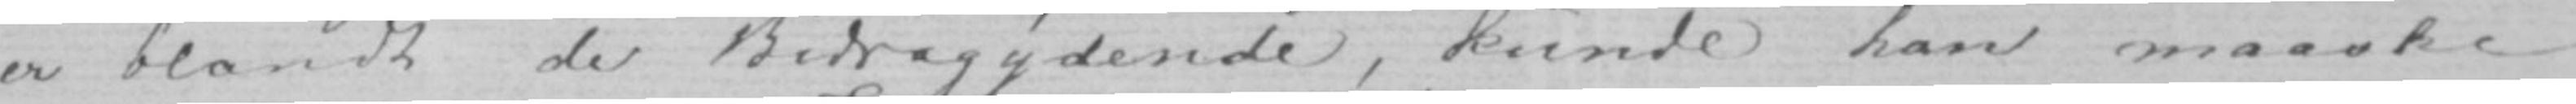er blandt de Bidragydende, kunde han maaske
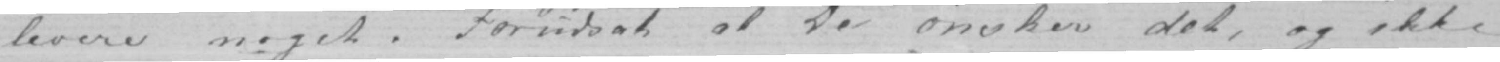levere noget. Forudsat at De ønsker det, og ikke
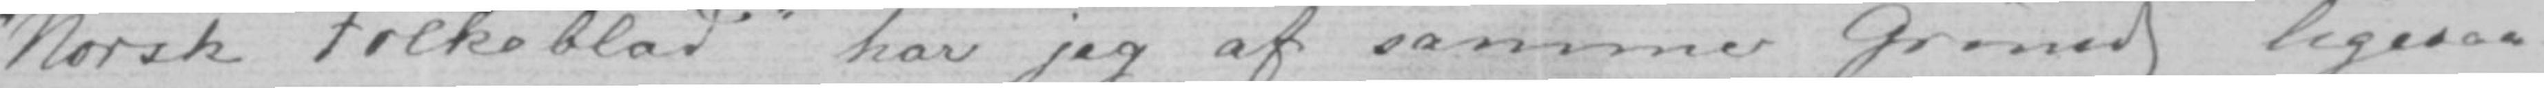«Norsk Folkeblad» har jeg af samme Grund ligesaa-
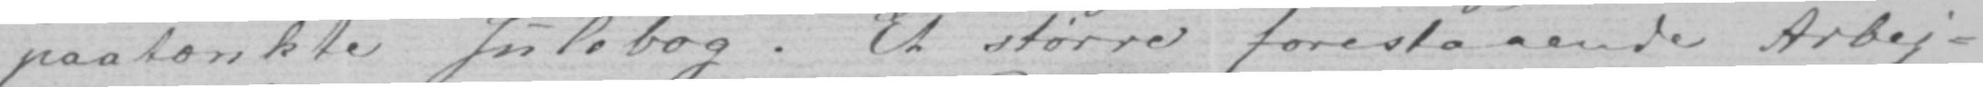paatænkte Julebog. Et større forestaaende Arbej-
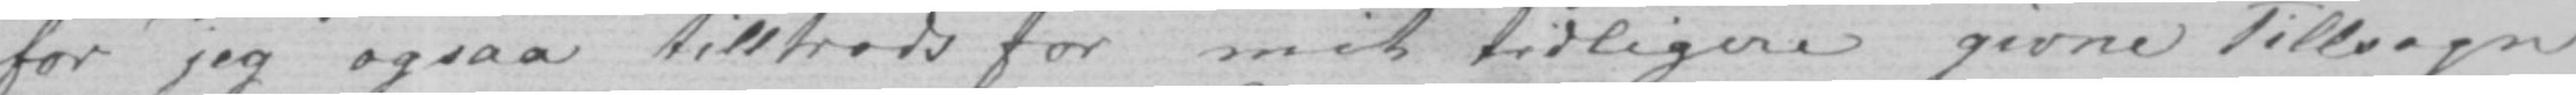for jeg ogsaa tilltrods for mit tidligere givne Tillsagn
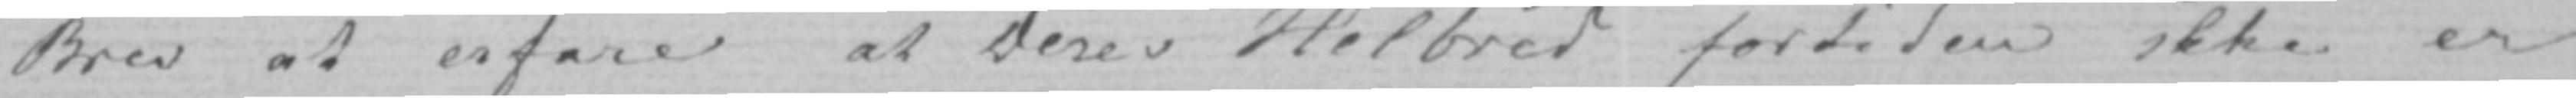Brev at erfare at Deres Helbred fortiden ikke er
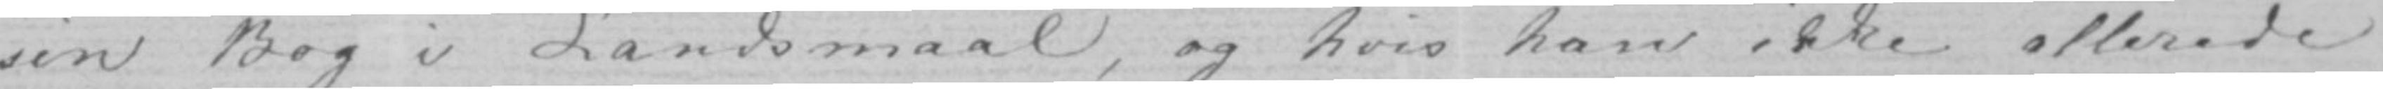sin Bog i Landsmaal, og hvis han ikke allerede
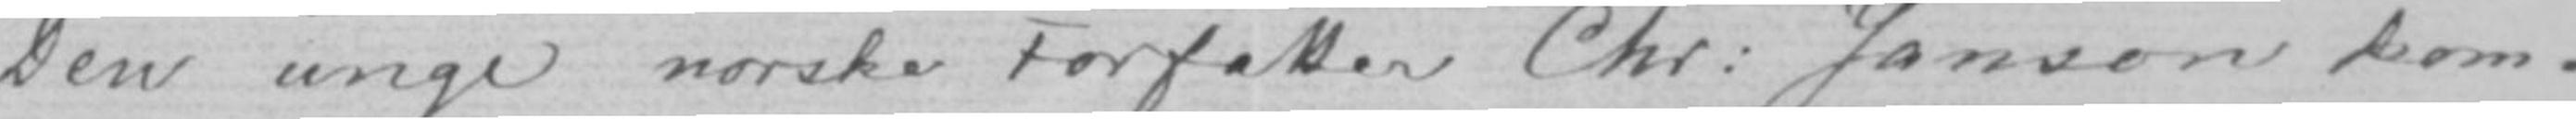Den unge norske Forfatter Chr: Janson kom-
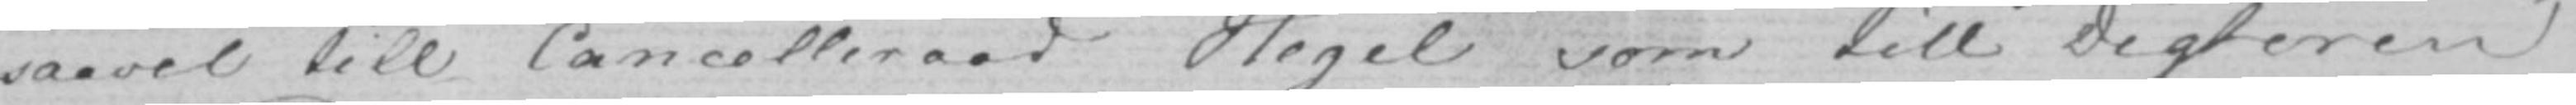saavel till Cancelliraad Hegel som till Digteren
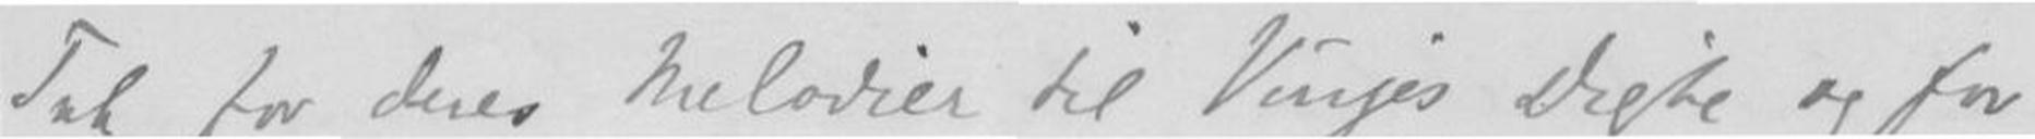Tak for deres Melodier til Vinjest Digte og for
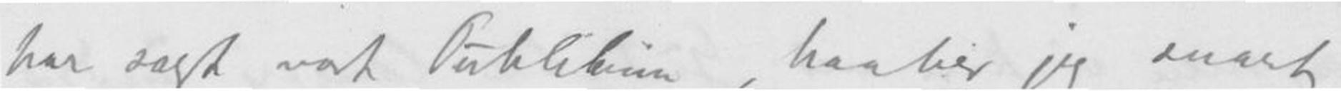har sagt vort Publikum, haaber jeg snart
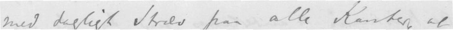med dagligt Stræb paa alle Kanter at
